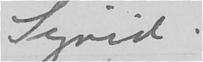din Sigrid
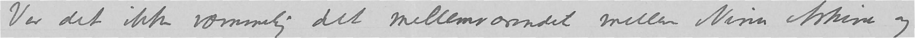Var det ikke væmmelig det mellemværendet mellem Nina Arkina og
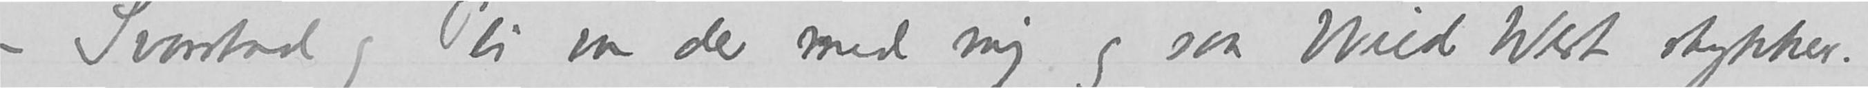Svarstad og Pei var der med mig og saa Wild West stykker.
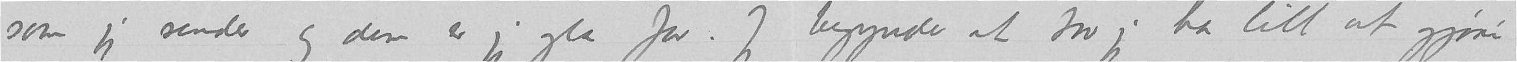som jeg sender og dem er jeg gla for.  Jeg begynder at tro jeg har litt at gjøre
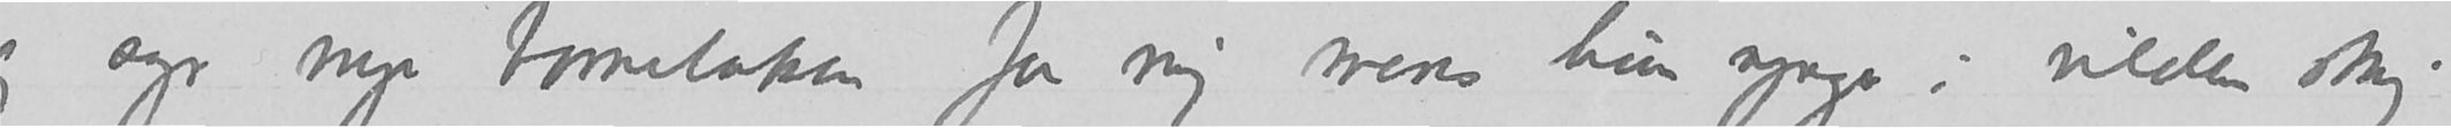og syr nye formlaken for mig mens hun synger i vilden sky.
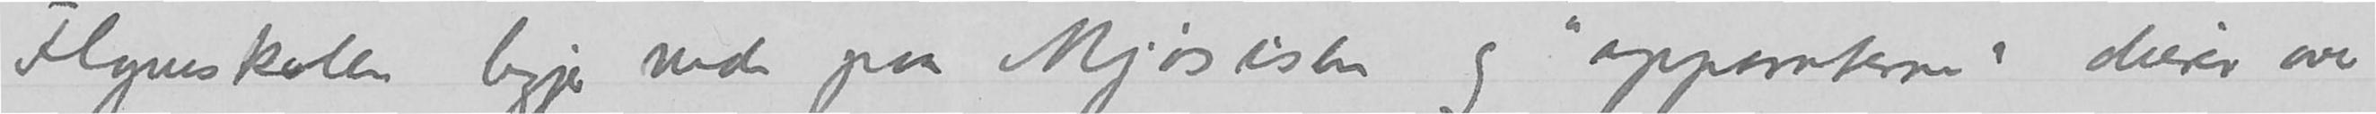Flyverskolen ligger nede paa Mjøsisen og «apparaterne» driver over
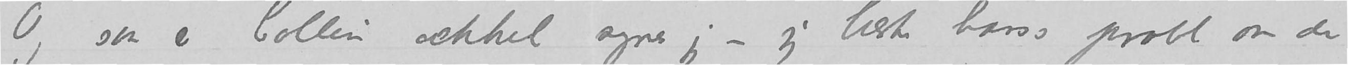Og saa er Collin ækkel synes jeg - jeg leste tross hans probl. om de
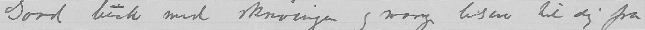Good luck med skrivingen og mange hilsener til dig fra
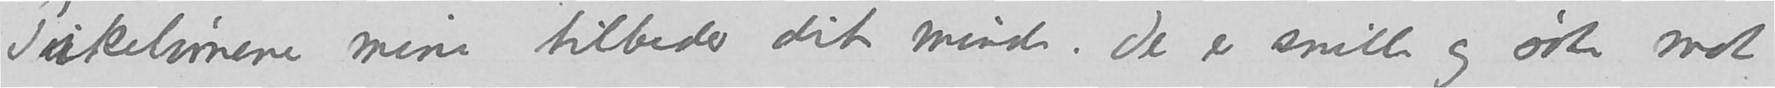Pikebørnene mine tilbeder dit minde. De er snille og søte mot
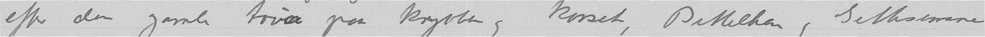efter den gamle trua paa krybben og korset, Betlehem og Gethsemane
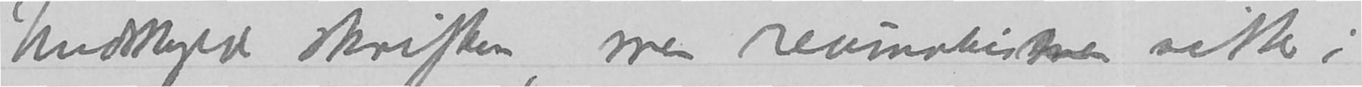Undskyld skriften, men reumatismen sitter i skulderbladene!
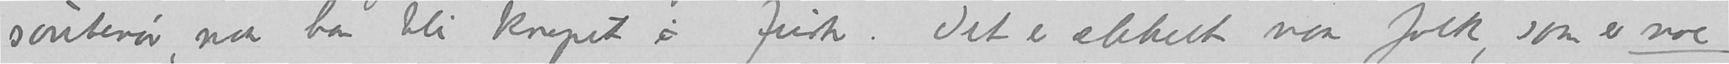souterøru/soutenør, naar han blir knepet i fusk. Det er ekkelt naar folk, som er, noe
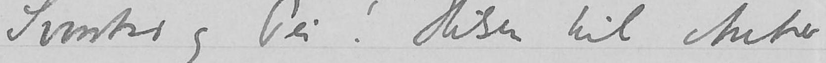Svarstad og Pei! Hilsen til Anker
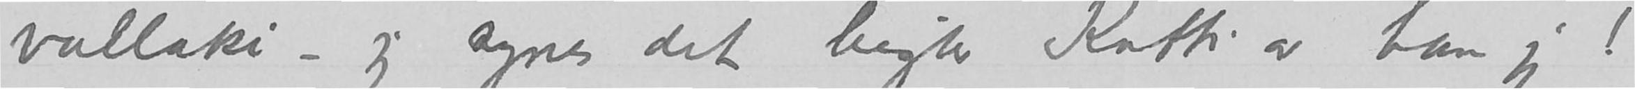vallaki - jeg synes det lugter Kaffi av ham jeg!
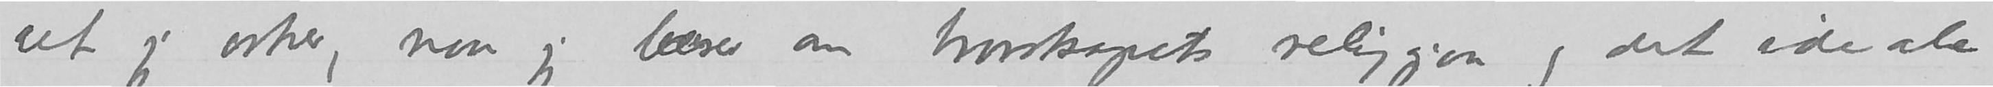alt jeg orker, naar jeg læser om brorskapets religion og det ideale
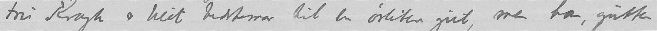Fru Krogh er blit bedstemor til en ørliten gut, men han, gutten
